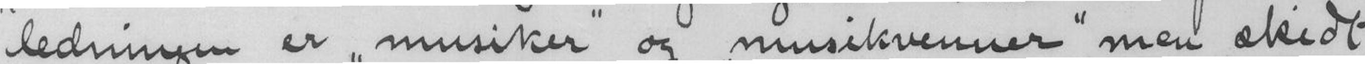ledningen er musiker og musikvenner men skidt
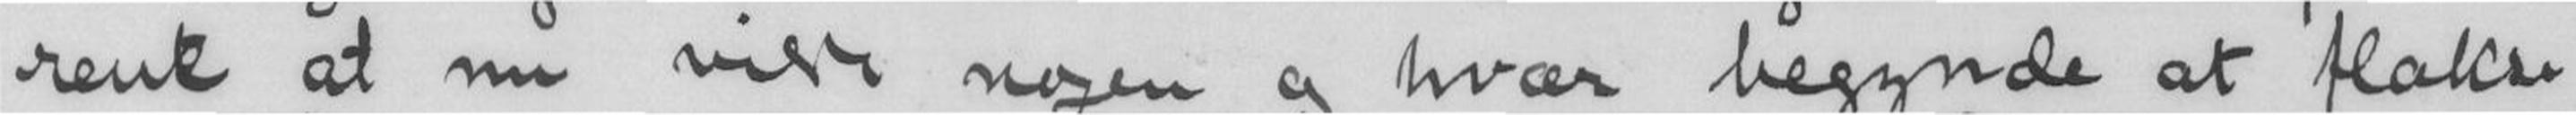rene at nu vilde nogen og hvær begynde at flakse
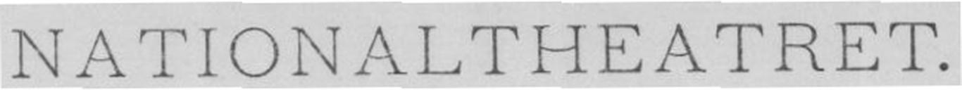NATIONALTHEATRET.
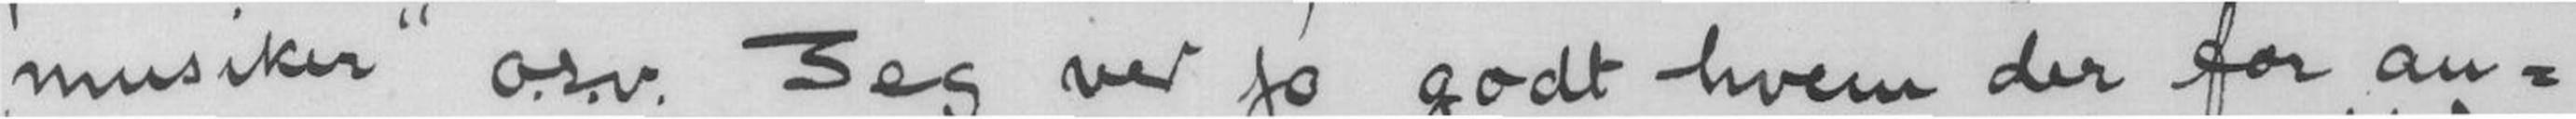musiker o.s.v. Jeg ved jo gpdt hvem der for an-
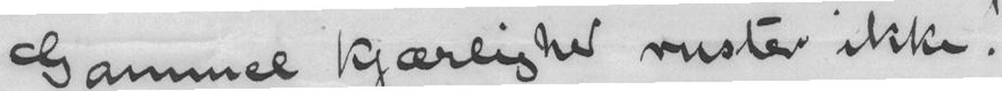Gammel kjærlighed ruster ikke!
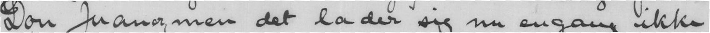Don Juan er, men det lader sig nu engang ikke
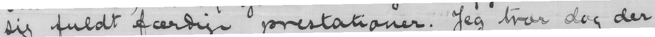sig fuldt færdige prestationer. Jeg tror dog der
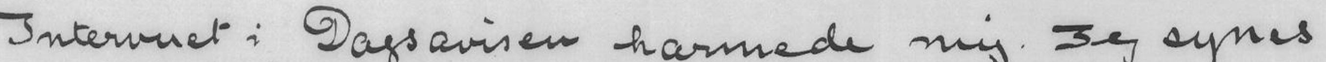Intervuet i Dagsavisen harmede mig. Jeg synes
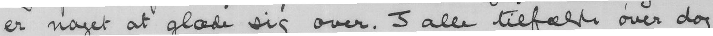er noget at glæde sig over. I alle tilfælde øver dog
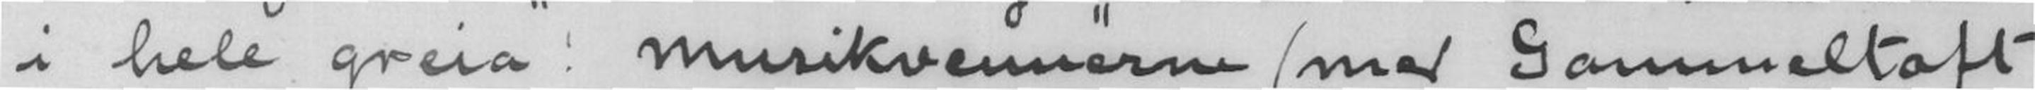i hele greia! Musikvennerne (med Gammeltoft
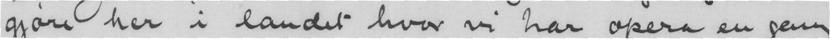gjøre her i landet hvor vi har opera en gang
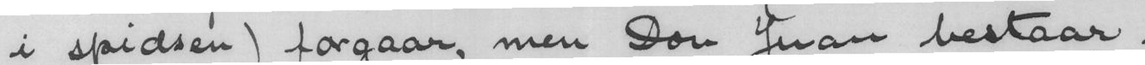i spidsen) forgaar, men Don Juan bestaar.
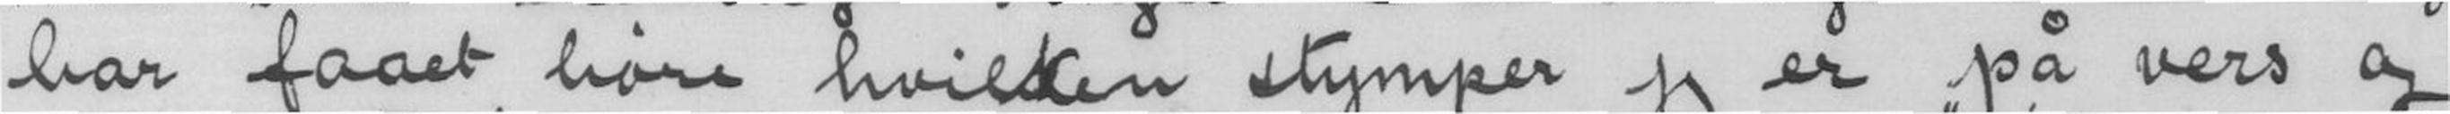har faaet høre hvilken stymper jeg er "på vers og
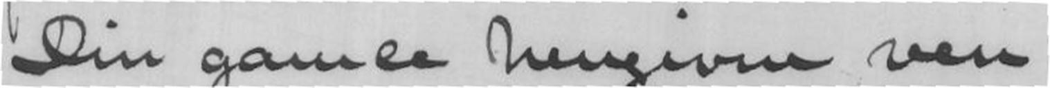Din gamle hengivne ven
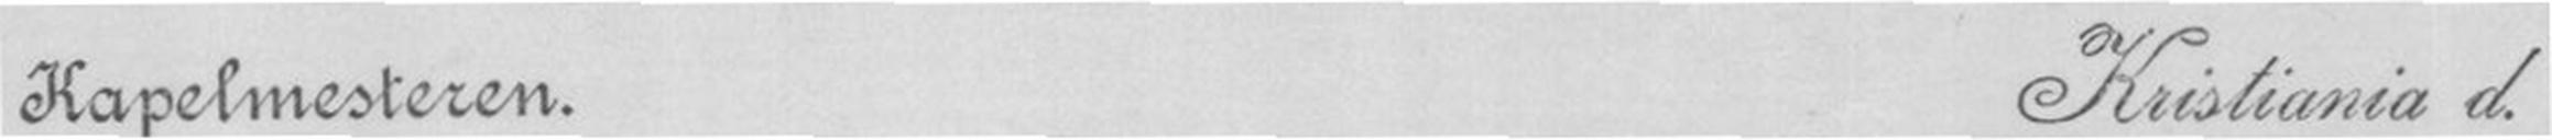Kapelmesteren. Kristiania d.
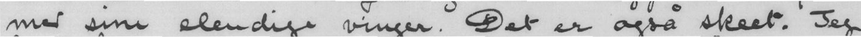med sine elendige vinger. Det er også skeet. Jeg
